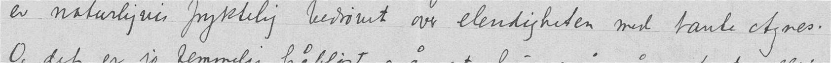er naturligvis fryktelig bedrøvet over elendigheten med tante Agnes.
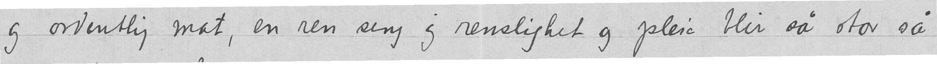og ordentlig mat, en ren seng og renslighet og pleie blir så stor så
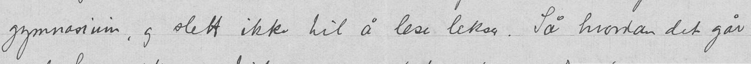gymnasium, og slett ikke til å lese lekser. Så hvordan det går
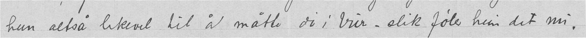hun altså likevel til å måtte dø i bur – slik føler hun det nu.
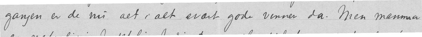gangen er de nu alt i alt svært gode venner da. Men mamma
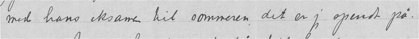med hans eksamen til sommeren, det er jeg spendt på.
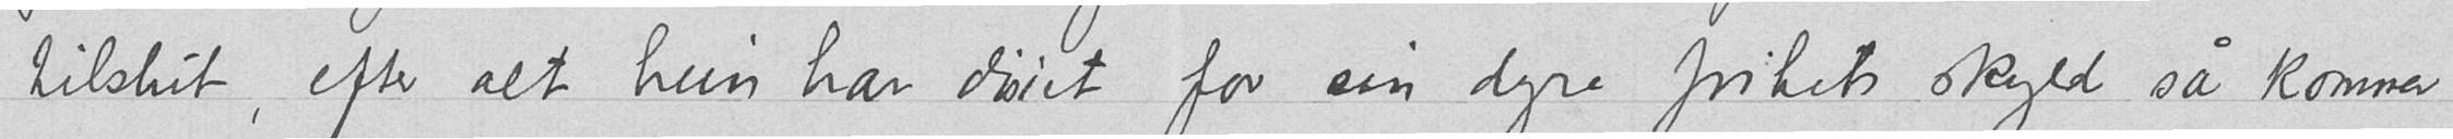tilslut, efter alt hun har døiet for sin dyre frihets skyld så kommer
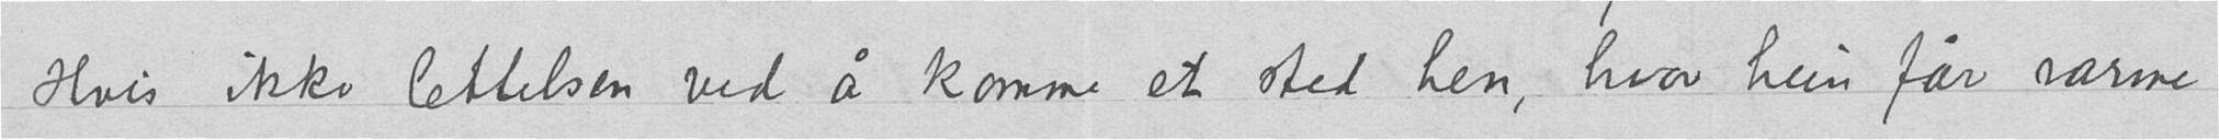Hvis ikke lettelsen ved å komme et sted hen, hvor hun får varme
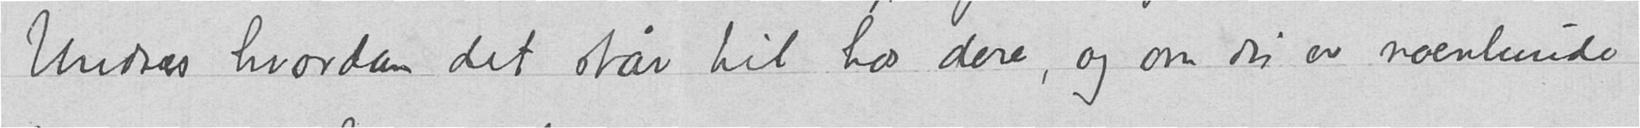Undres hvordan det står til hos dere, og om du er noenlunde
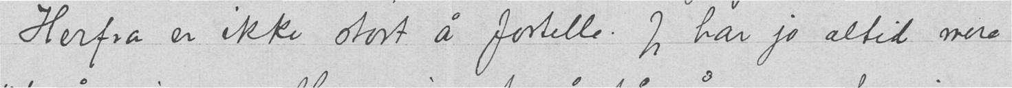Herfra er ikke stort å fortelle. Jeg har jo altid mere
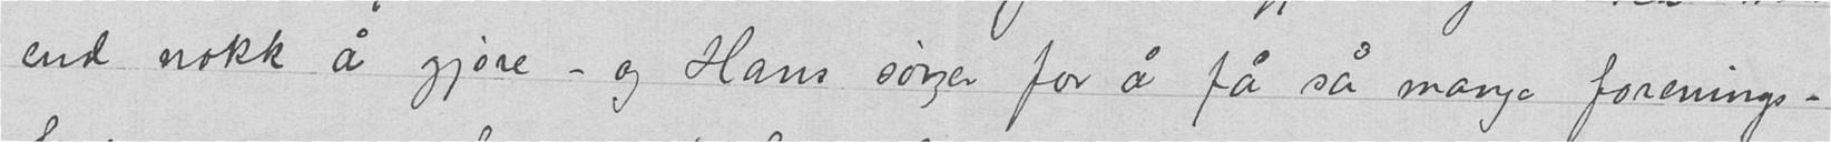end nokk å gjøre – og Hans sørger for å få så mange forenings-
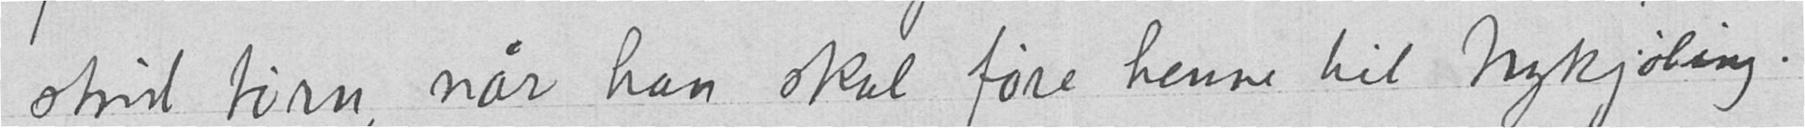strid tørn, når han skal føre henne til Nykjøbing.
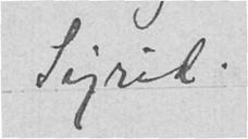Sigrid.
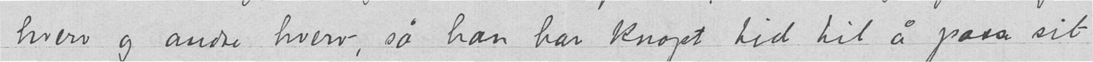hverv og andre hverv, så han har knapt tid til å passe sit
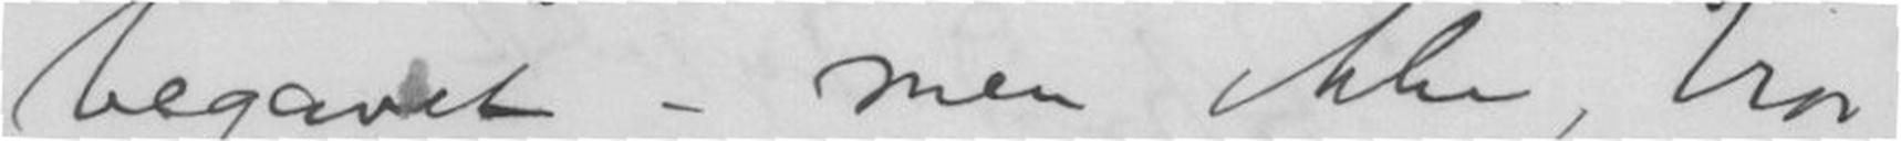begavet - men ikke, tror
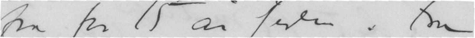fra for 15 år siden. Fru
